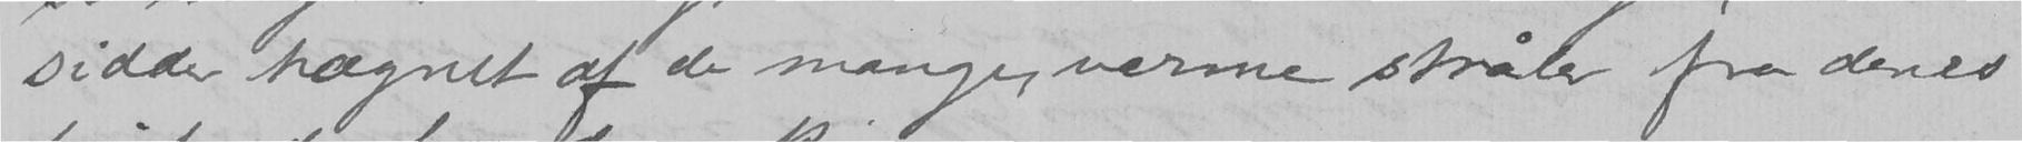sidder hægnet af de mange varme stråle fra deres
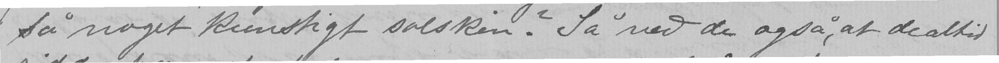så noget kunstigt solskin? Så ved de også, at de altid
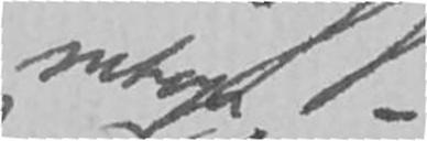netop
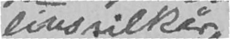livsvilkår
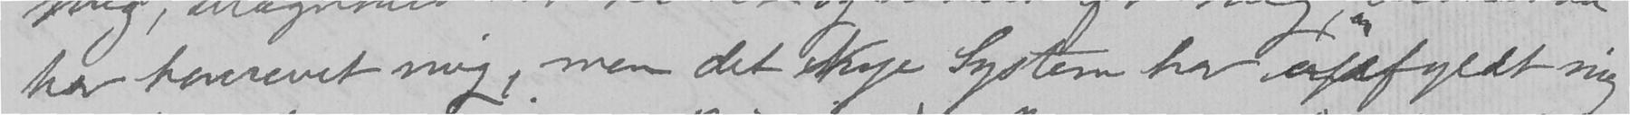har henrevet mig, men det Nye System har overfyldt mig
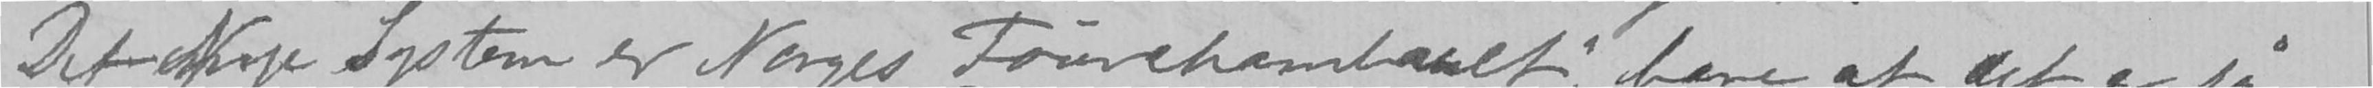Det Nye System er Norges Fourchambault; bare at det er så
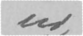ud
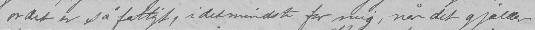ordet er så fattigt, i det mindste for mig, når det gjelder
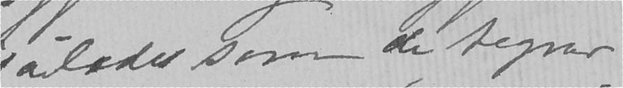således som de tegner
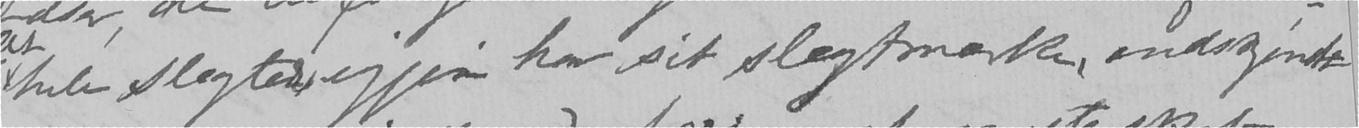hele slægter igjen har sit slægtmærke, endskjønt
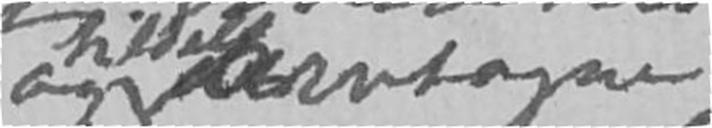og tildels arvtagne
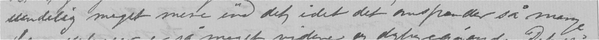uendelig meget mere end det, idet det omspænder så mange
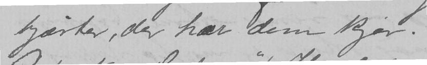hjærter, der har dem kjær.
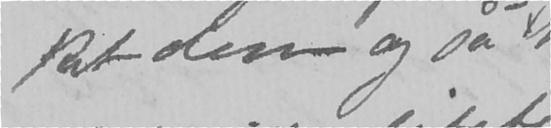ket dem og så
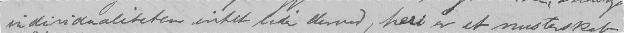individualiteten intet lider derved, her er et mesterskab
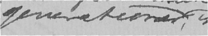generationers
